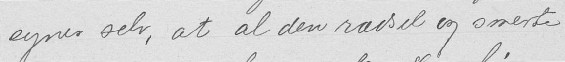synes selv, at al den rædsel og smerte
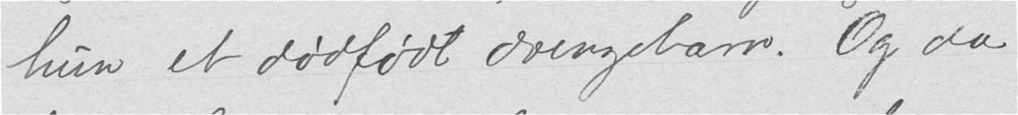hun et dødfødt drengebarn. Og da
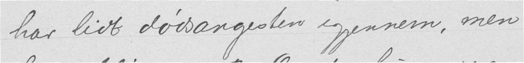har lidt dødsangesten igjennem, men
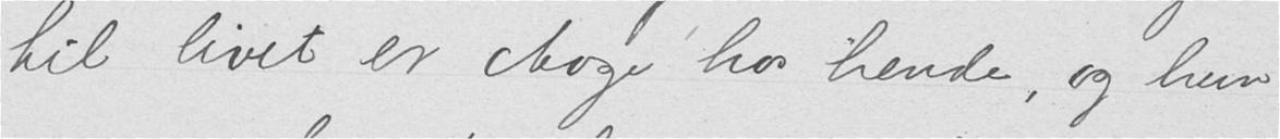til livet er Aage hos hende, og hun
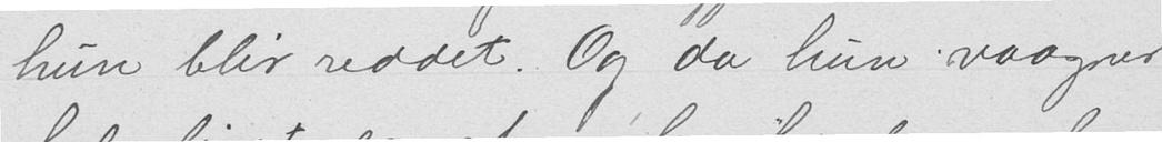hun blir reddet. Og da hun vaagner
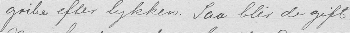gribe efter lykken. Saa blir de gift.
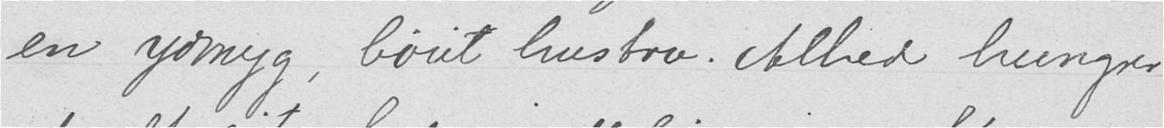en ydmyg, bøiet hustru. Alhed hungrer
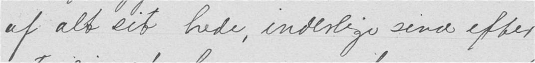af alt sit hede, inderlige sind efter
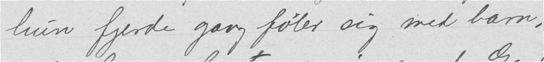hun fjerde gang føler sig med barn,
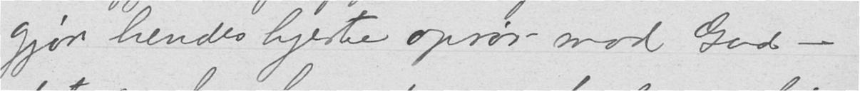gjør hendes hjerte oprør mod Gud –
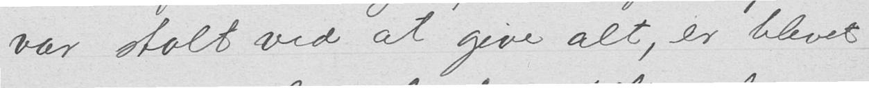var stolt ved at give alt, er blevet
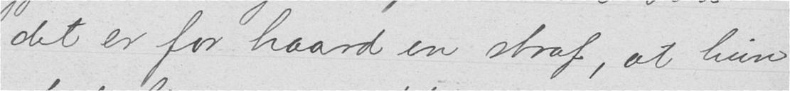det er for haard en straf, at hun
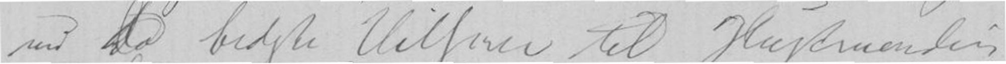nu de bedste Hilsener til Hustruen din
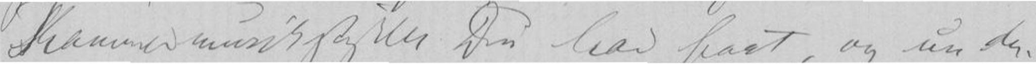Kammermusikstykker Du har faat, og under-
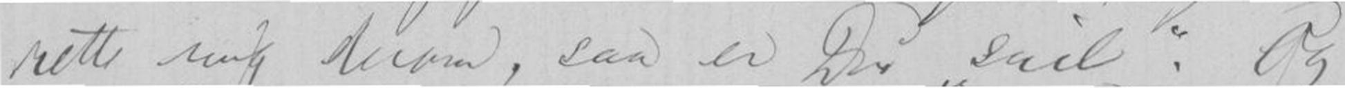rette mig derom, saa er Du snil. Og
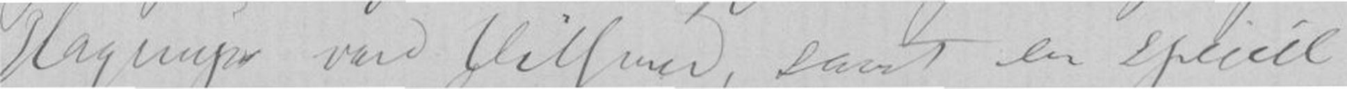Hagerups vore Hilsener, samt en speciel
